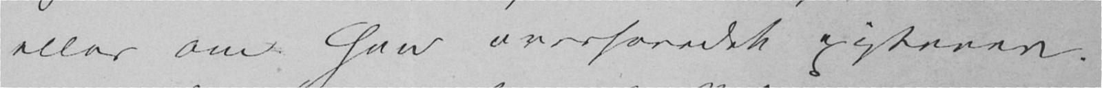eller om han overhovedet existerer.
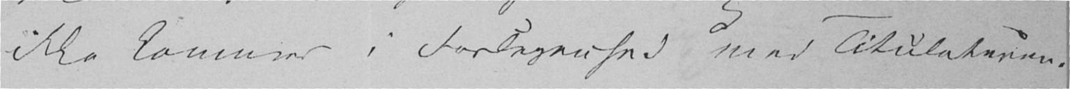ikke kommer i Forlegenhed med Titulaturen.
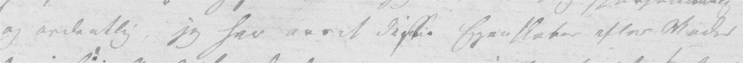og ordentlig, jeg har arvet disse Egenskaber efter Moder
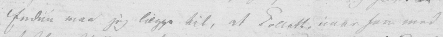Endnu maa jeg lægge til, at Collett, naar han med
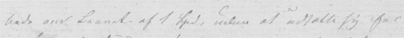bede om Laanet af 1 Spd, uden at udsætte sig for
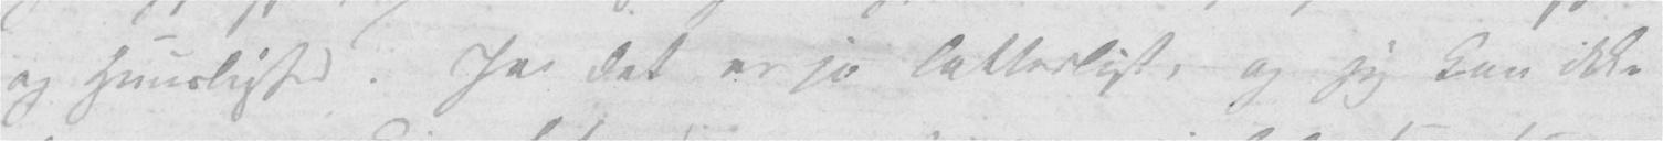og Huuslighed. Ja det er jo latterligt, og jeg kan ikke
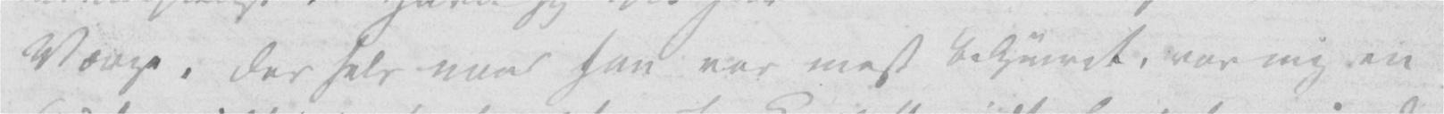Værge, der selv naar han var mest bekymret, var mig en
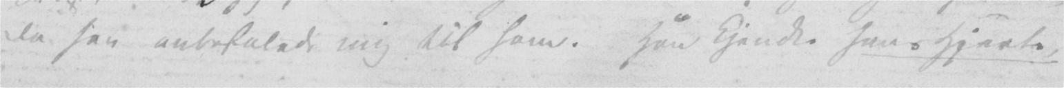da han anbefalede mig til ham. Han kjendte hans Hjerte,
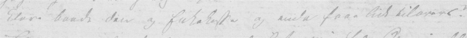klare baade den og Enkekasse og enda faae lidt tilovers?
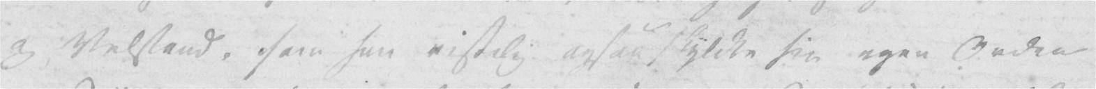og Velstand, som han visselig ogsaa skyldte sin egen Orden
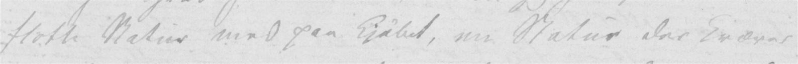flotte Natur med paa Kjøbet, en Natur der kræver
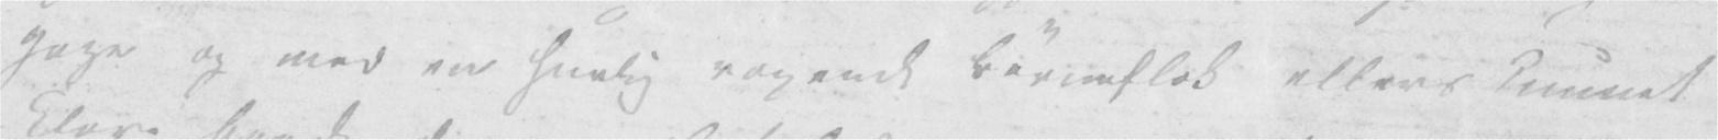Gage og med en hurtig voxende Børneflok ellers kunnet
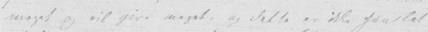meget og vil give meget, og dette er ikke saa let
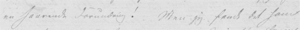en saarende Forundring! Men jeg fandt det som
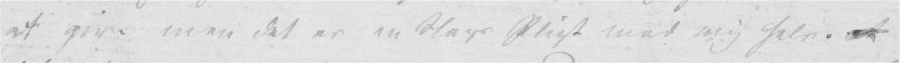at give men det er en Slags Pligt mod mig selv. et
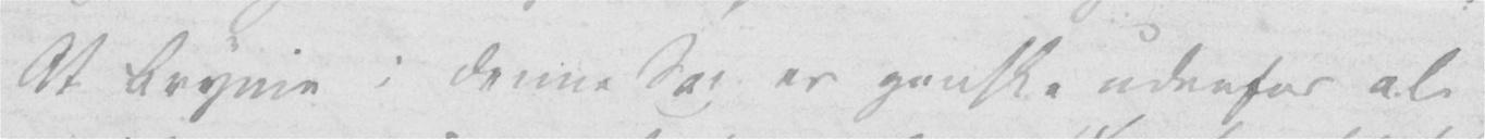At Brynie i denne Sag er ganske udenfor al
